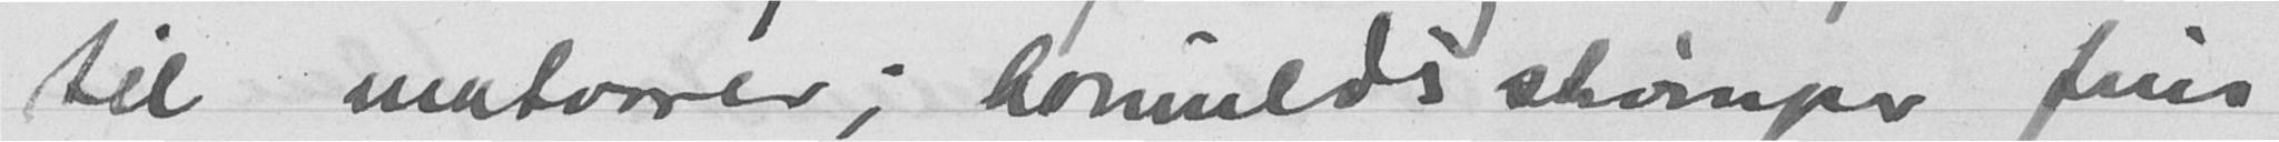til matvarer; bomulds strømper fins
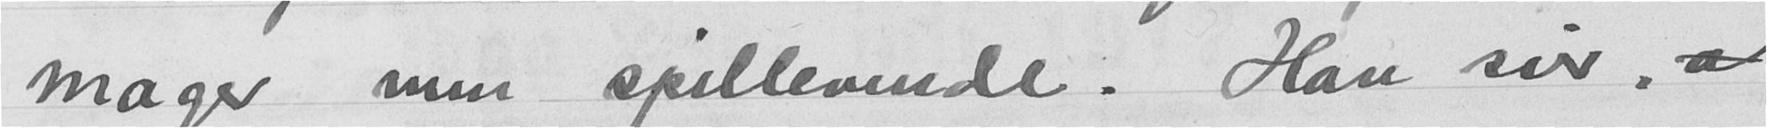mager men spillevende. Han sir, a
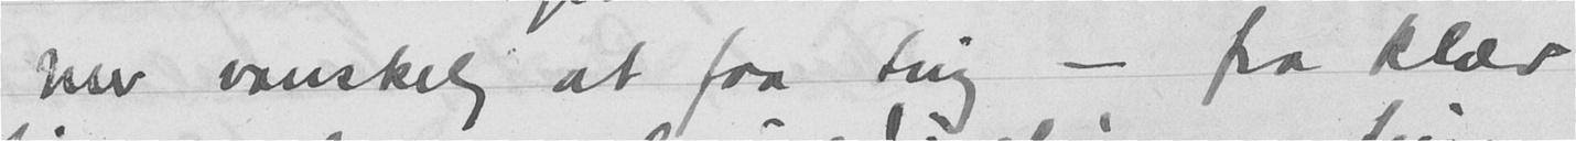mer vanskelig at faa ting - fra klær
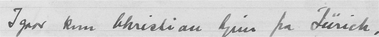Igaar kom Christian hjem fra Zürich,
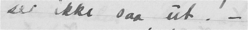ser ikke saa ut. -
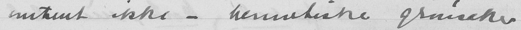omtrent ikke - hermetiske grønsaker
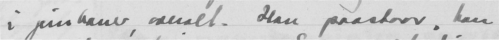i jernbaner, overalt. Han paastaar, han
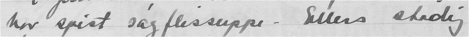har spist sagflissuppe. Ellers stadig
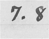7.8
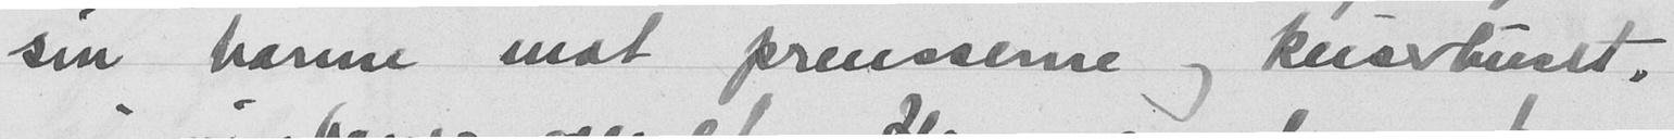sin harme mot preusserne og keiserhuset,
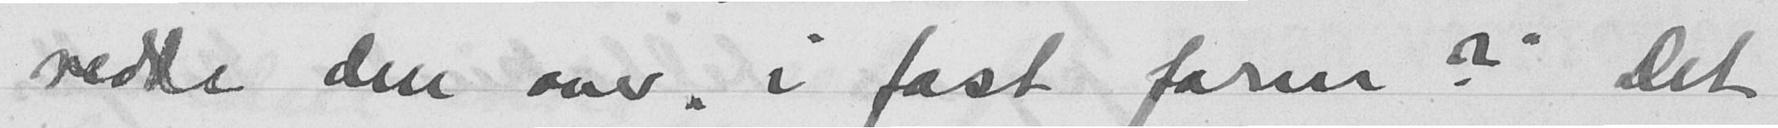redde den over "i fast form?" Det
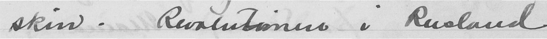skin. Revolutionen i Rusland
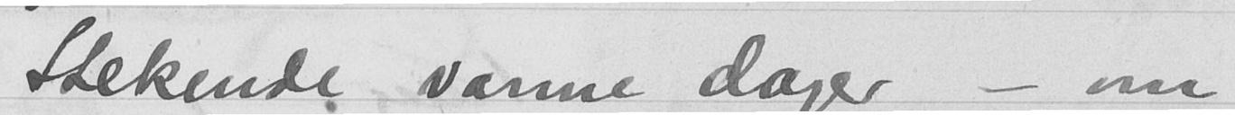Stekende varme dager - om
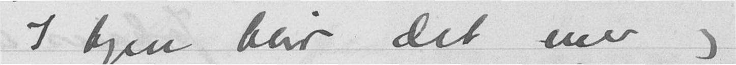I byen blir det mer og
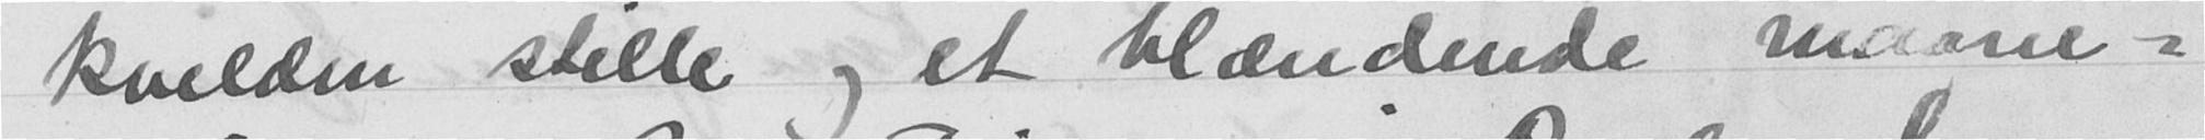kvelden stille og et blændende maane-
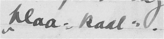"blaa-kaal".
